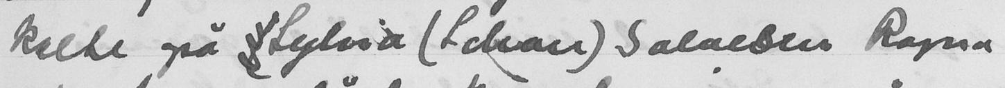kalte ogsåå Sylvia (Schon) Salaeber Ragna
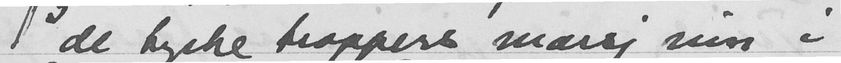de tyske troppers marsj inn i
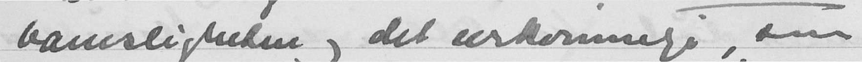barnsligheten og det urkvinnelige, som
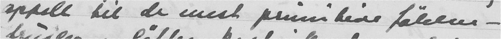appell til de mest primitive følelser -
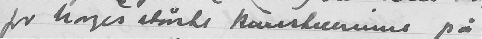for Norges største kunstnerinne på
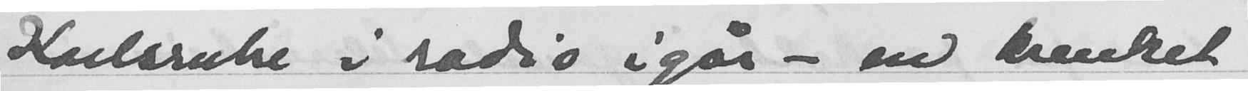Karlsruhe i radio igår - en krenket
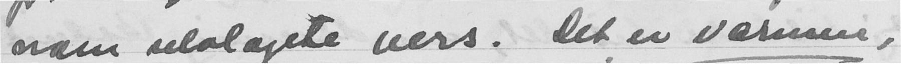noen selvlagete vers. Det er varmen,
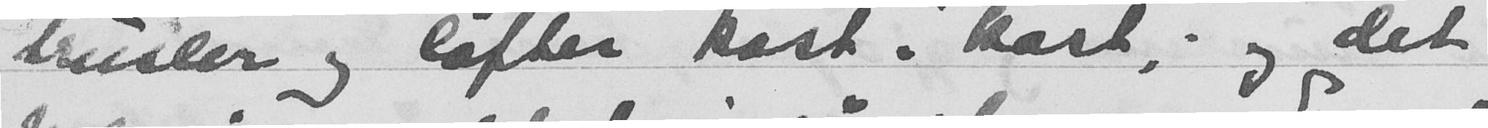bruster og løfter kast i kast; og det
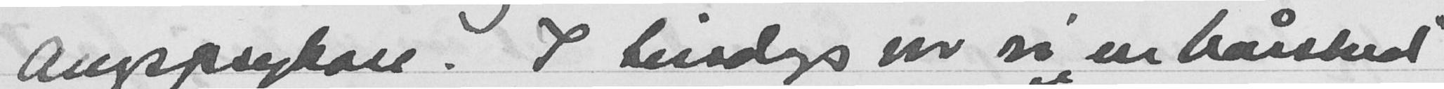angepspsykose. I tirsdags var vi "en hårsbred"
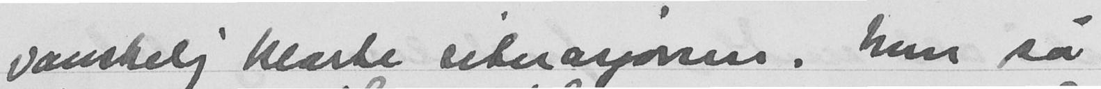vanskelig klarte situasjonen. Men så
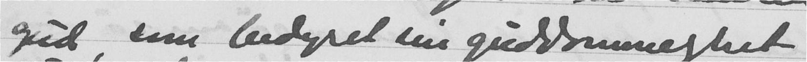gud, som bedyret sin guddommelighet
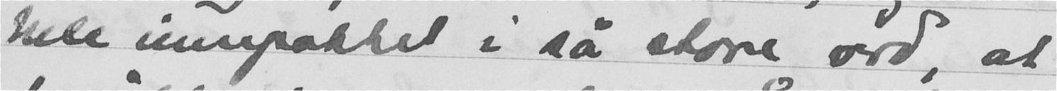hele innpakket i så store ord, at
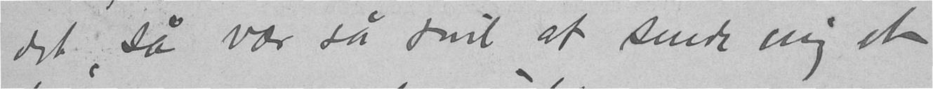det, så vær så snil at sende mig et
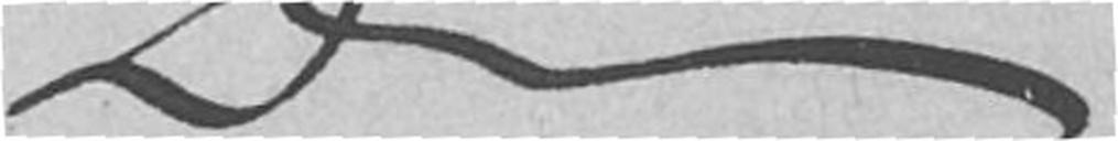Din
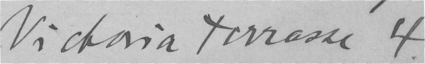Victoria Terrasse 4.
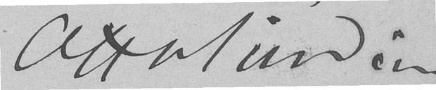Otto Sinding
